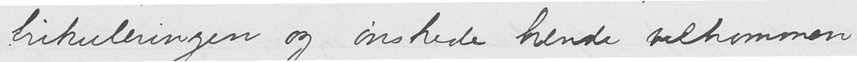trikuleringen og ønskede henne velkommen
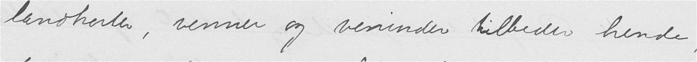landkarter, venner og veninder tilbeder hende,
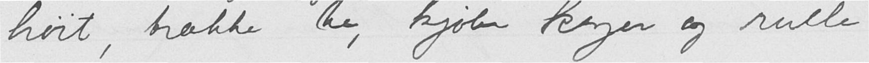høit, trække the, kjøbe kager og rulle
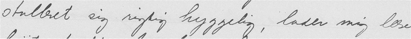stalleret sig rigtig hyggelig, lader mig læse
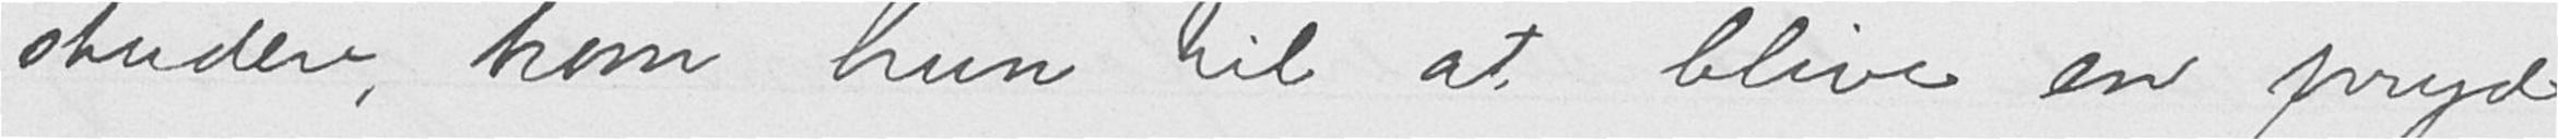studere, kom hun til at blive en pryd
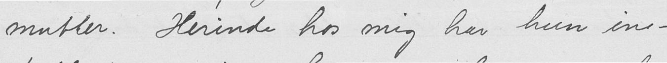mutter. Herinde hos mig har hun in-
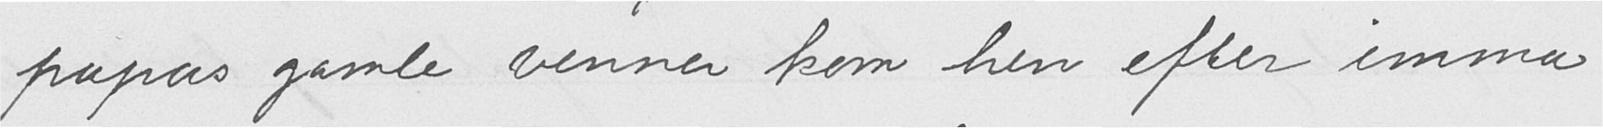papas gamle venner kom hen efter imma-
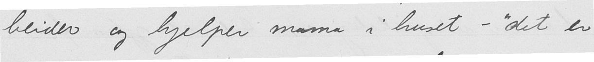beider og hjelper mama i huset - "det er
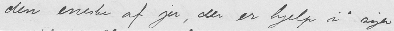den eneste af jer, der er hjelp i" siger
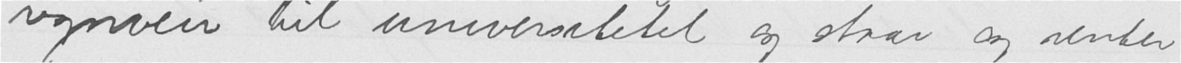regnveir til universitetet og staar og venter
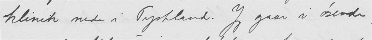klinik nede i Tyskland. Jeg gaar i øsende
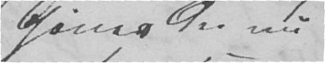Gives der nu
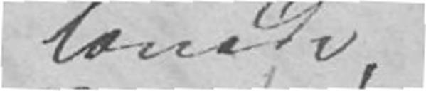lovede,
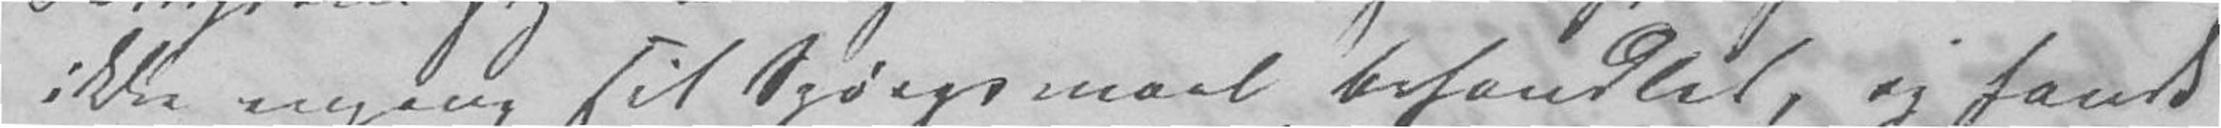ikke engang sit Spørgsmaal behandlet, og fandt
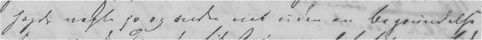sagde nogle jo og andre nei uden en Begrundelse
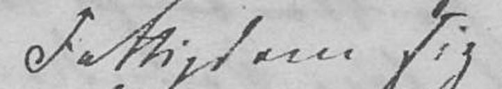Fattigdom sig
